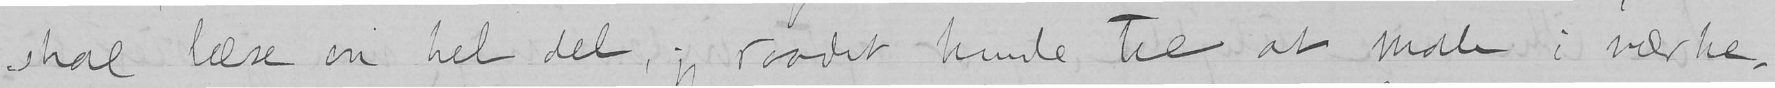skal læse en hel del, jeg raadet hende til at male i nærhe-
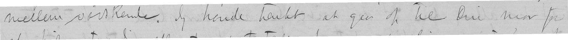mellem sødskende. Jeg havde tænkt at gaa op til Din mor for
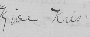Kjære Kris!
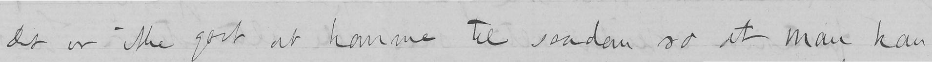Det er ikke godt at komme til saadan ro at man kan
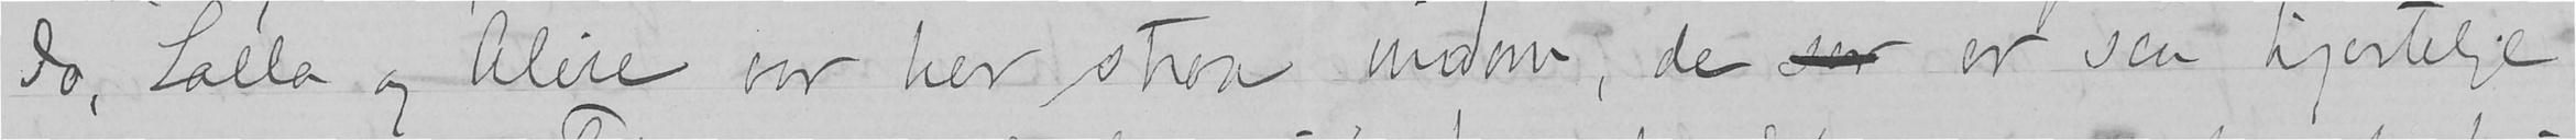Jo, Lalla og Alice var her strax indom, de ser er saa hjertelige
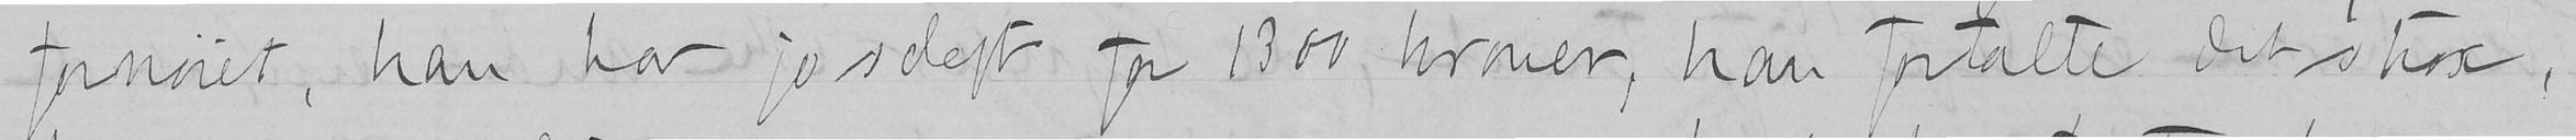fornøiet, han har jo solgt for 1300 kroner, han fortalte det strax,
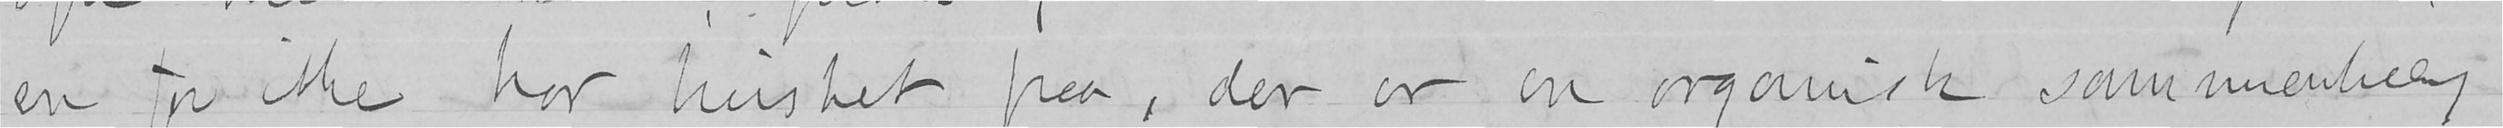en før ikke har husket paa, der er en organisk sammenhæng
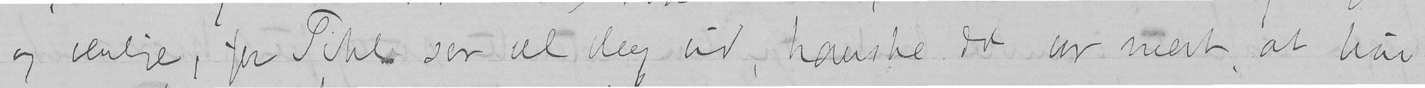og venlige, fru Pihl ser vel bleg ud, kanske det var mest, at hun
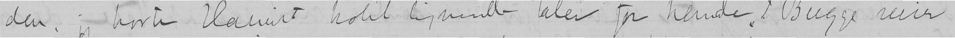den, jeg hørte Harriet holdt lignende taler for hende. J. Bugge reiser
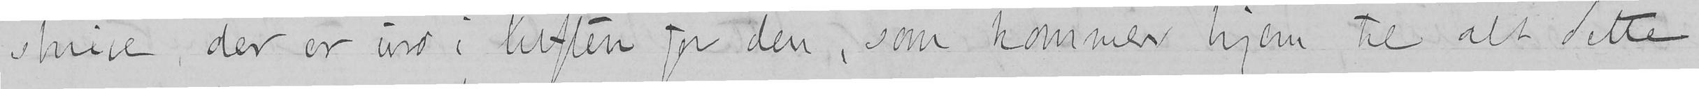skrive, der er uro i luften for den, som kommer hjem til alt dette
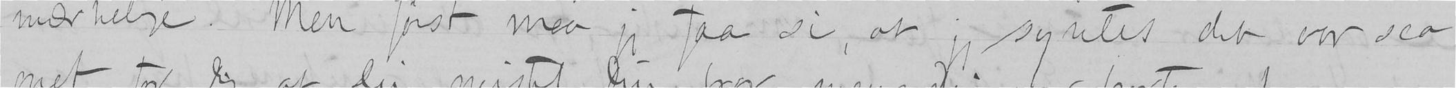mærkelige. Men først maa jeg faa si, at jeg syntes det var saa
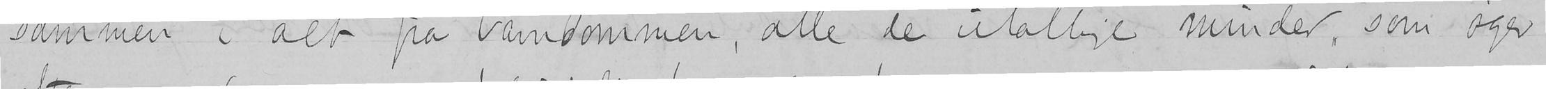sammen i alt fra barndommen, alle de utallige minder, som øger
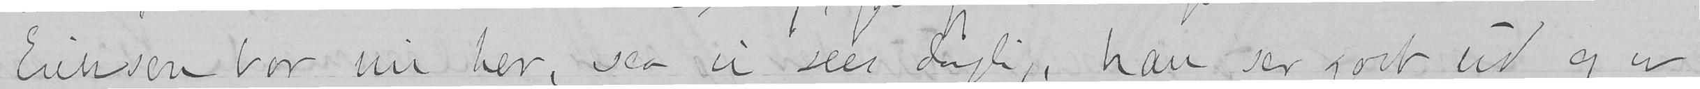Eriksen bor nu her, saa vi sees daglig, han ser godt ud og er
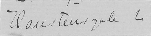Hanstensgate 2
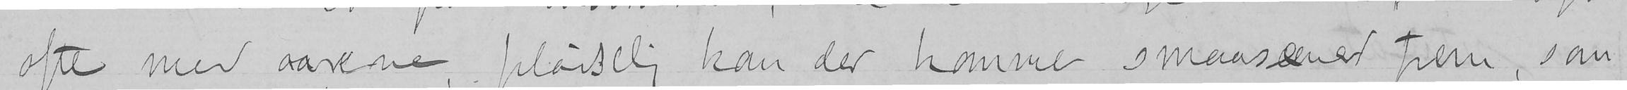ofte med aarene, pludselig kan der kommer smaascener frem, som
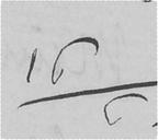16/6
